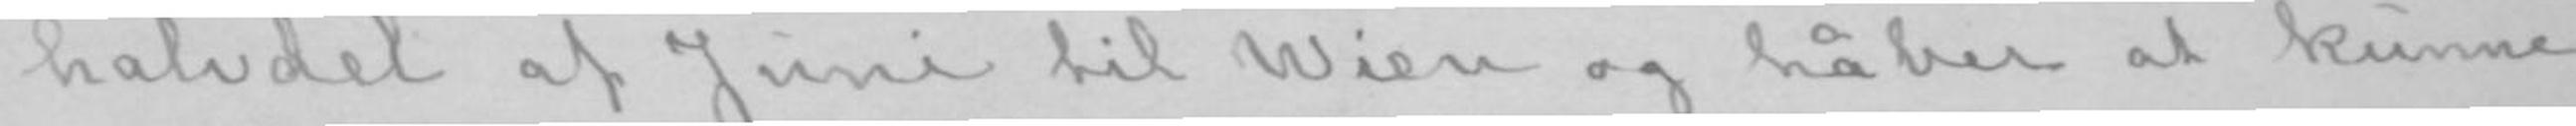halvdel af Juni til Wien og håber at kunne
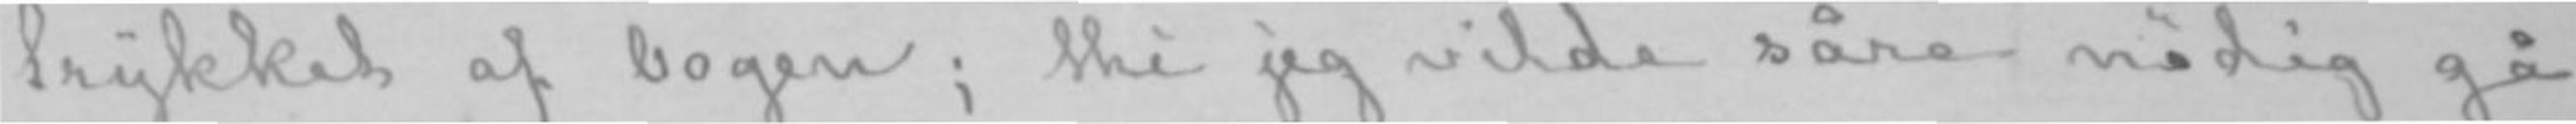trykket af bogen; thi jeg vilde såre nødig gå
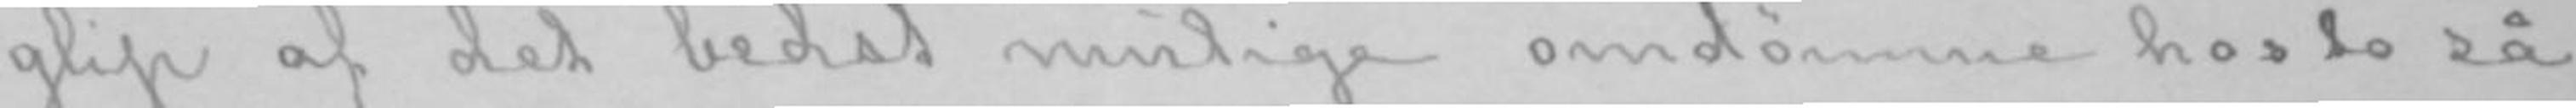glip af det bedst mulige omdømme hos to så
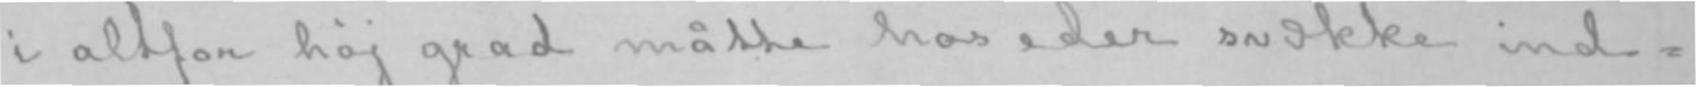i altfor høj grad måtte hos eder svække ind-
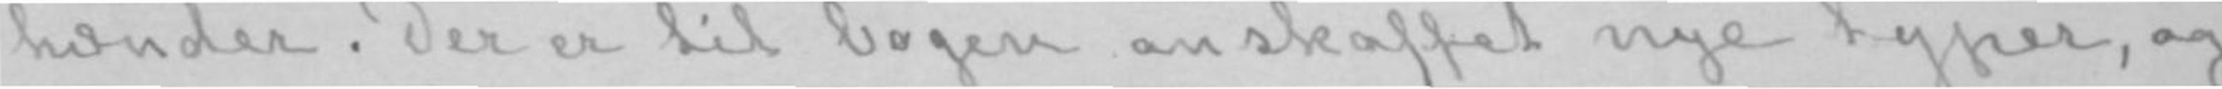hænder. Der er til bogen anskaffet nye typer, og
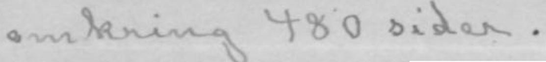omkring 480 sider.
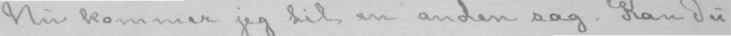Nu kommer jeg til en anden sag. Kan Du
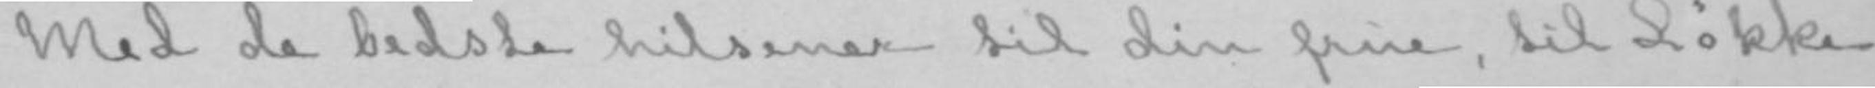Med de bedste hilsener til din frue, til Løkke
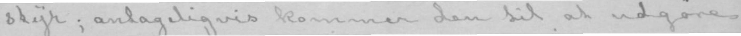styr; antageligvis kommer den til at udgøre
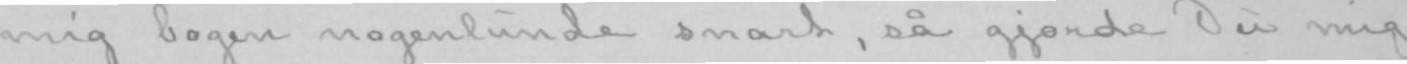mig bogen nogenlunde snart, så gjorde Du mig
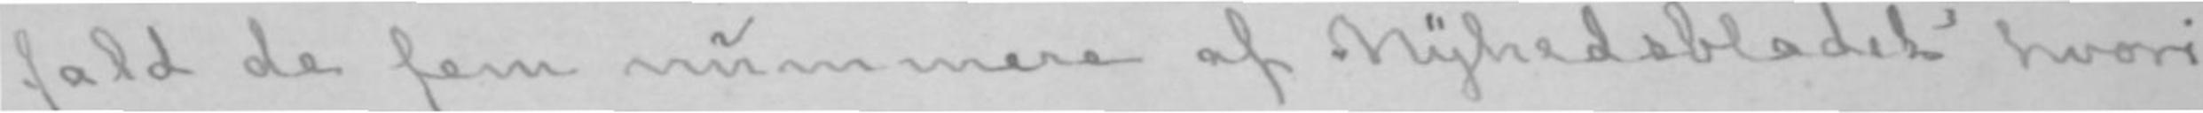fald de fem nummere af «Nyhedsbladet» hvori
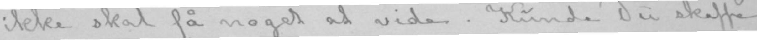ikke skal få noget at vide. Kunde Du skaffe
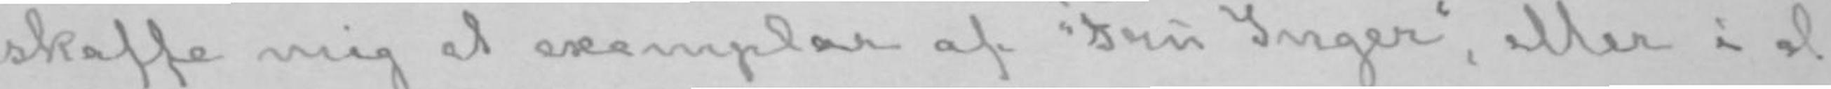skaffe mig et exemplar af «Fru Inger», eller i al
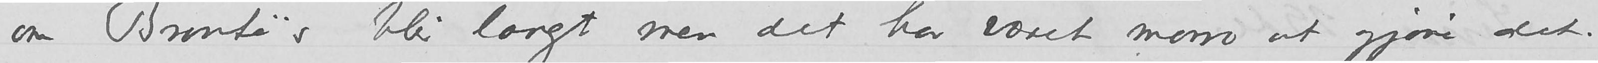om Brontës blir langt men det har været morro at gjøre det.
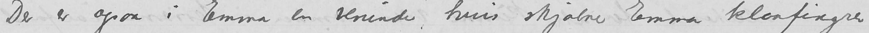Der er ogsaa i Emma en kvinde hvis skjæbne Emma klaafingrer
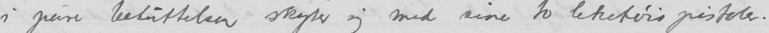i pure betuttelsen skyter sig med sine leketøispistoler.
